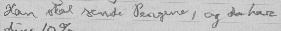Han skal sende Pengene, og da har
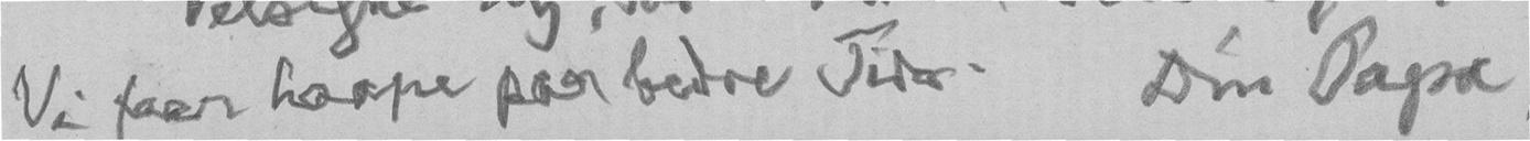Vi faar haape paa bedre Tider. Din Papa
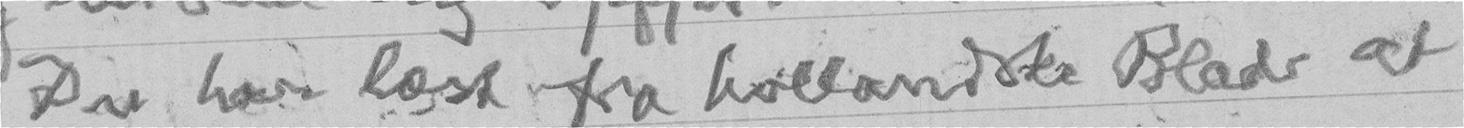Du har læst fra hollandske Bladr at
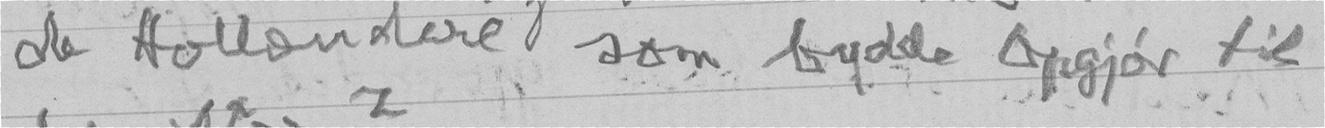de Hollændere som bydde Opgjør til
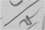/v
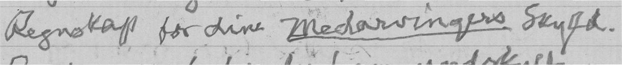Regnskap for dine Medarvingers Skyld.
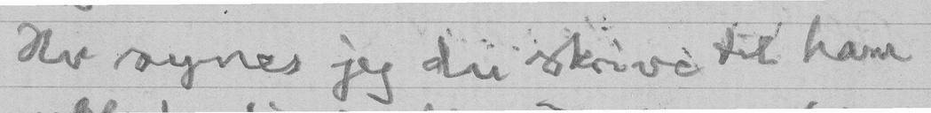Nu synes jeg du skrive til ham
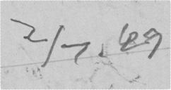2/7. 49
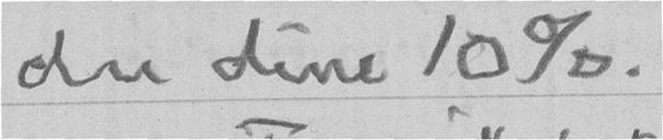du dine 10%.
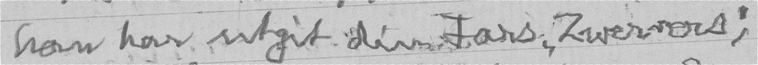han har utgit din Fars "Zwervers",
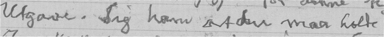Utgave. Sig ham at du maa holde
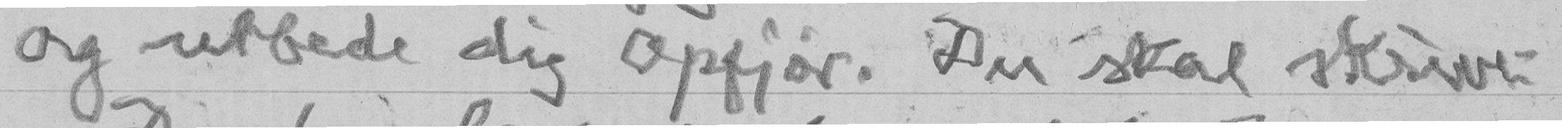og utbede dig Opgjør. Du skal skrive:
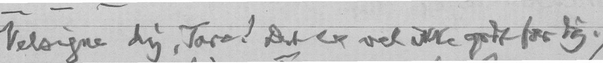Velsigne dig, Tore! Det er vel ikke godt for dig.
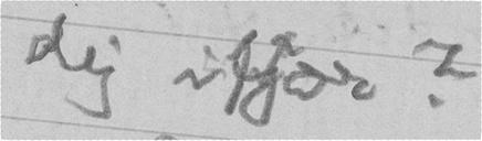dig ifjor?
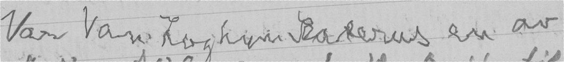Var Van Loghum Slaterus en av
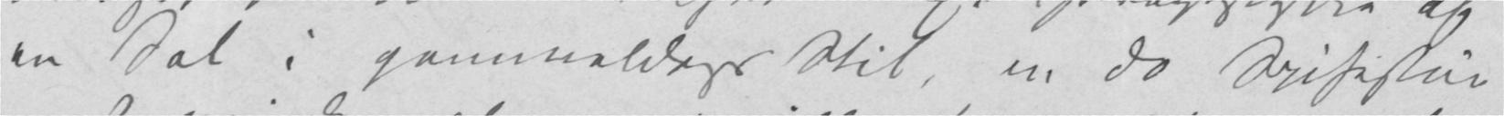en Sal i gammeldags Stil, en do Spisestue
